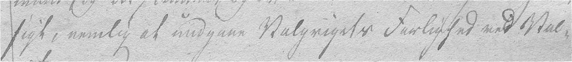sigt, nemlig at undgaae Valgrigets Farlighed ved Val-
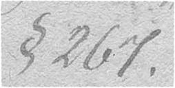§ 261.
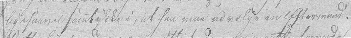ligesaavel samtykke i, at han maa udvælge en Eftermand.
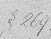§ 264.
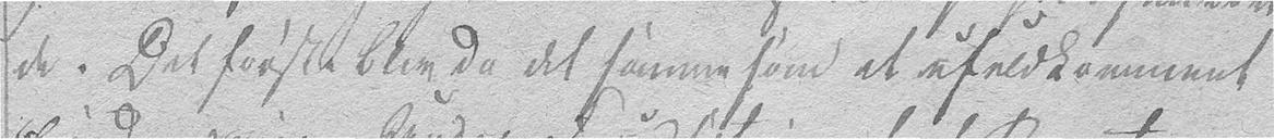de. Det første blev da det samme som et ufuldkomment
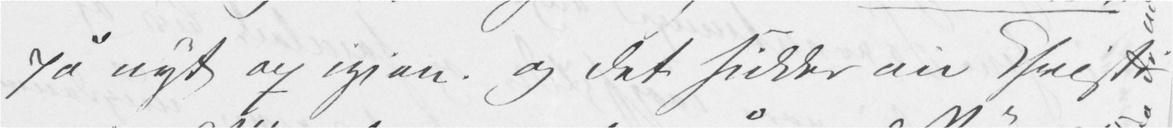på nyt op igjen. og det sidder nu Christi-
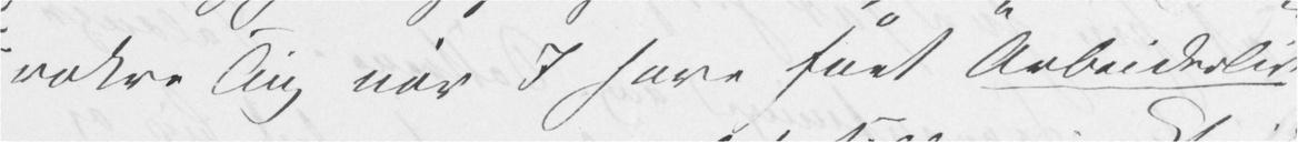vakre Ting når I have fået "Arbeiderliv
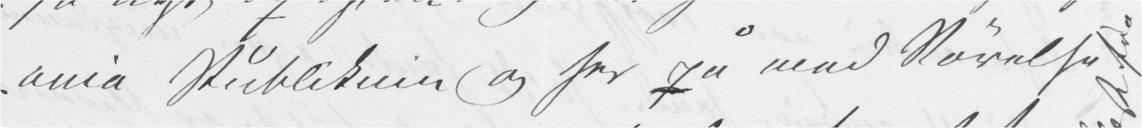ania Publikum og ser på med Rørelse.
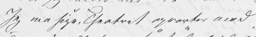Jeg ma sige, Theatret opvarter med
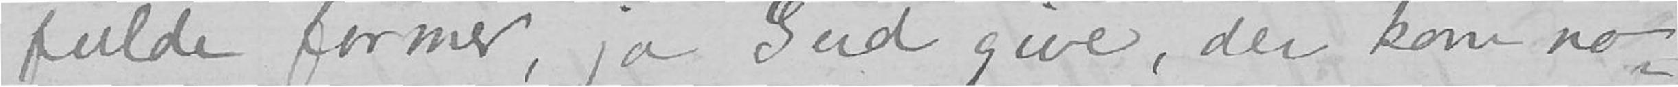fulde former, ja Gud give, der kom no-
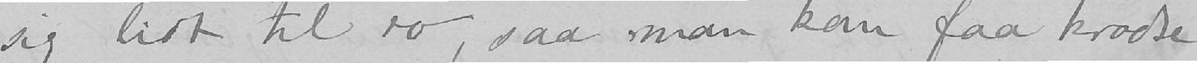sig lidt til ro, saa man kan faa kradse
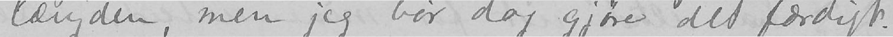længden, men jeg bør dog gjøre det færdigt.
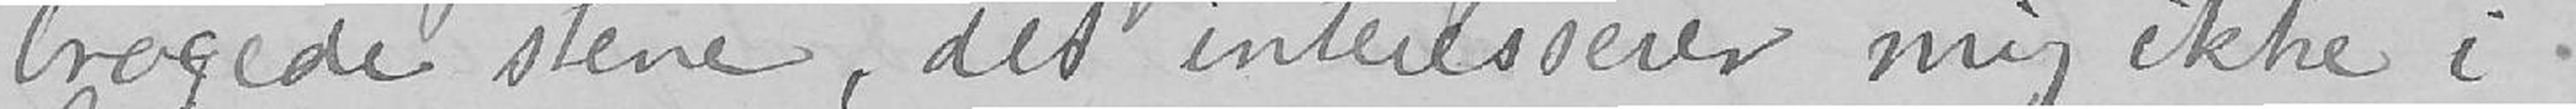brogede stene, det interesserer mig ikke i
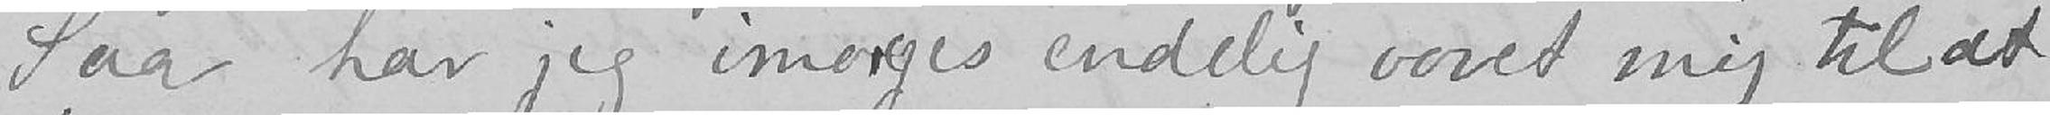Saa har jeg imorges endelig vovet mig til at
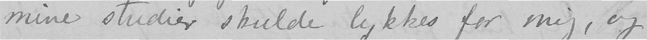mine studier skulde lykkes for mig, og
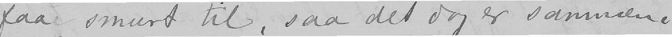faa smurt til, saa det dog er sammen i
